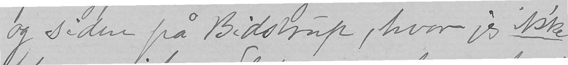og siden på Bidstrup, hvor jeg ikke
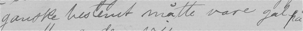ganske bestemt måtte være gal på
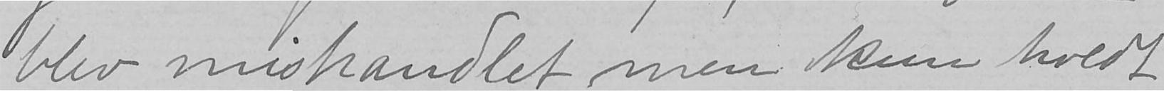blev mishandlet men kun holdt
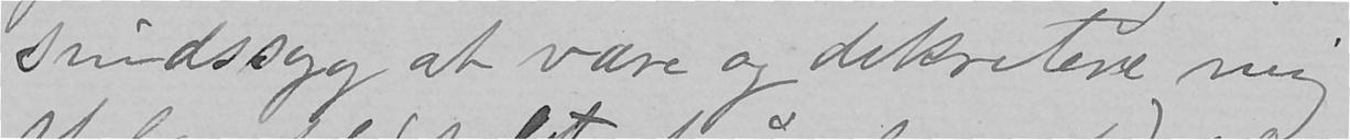sindssyg at være og dekretere mig
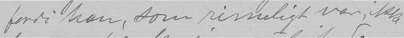fordi han, som rimeligt var, ikke
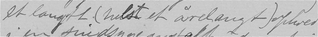et langt (helst et årelangt) ophold
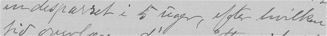indespærret i 5 uger, efter hvilken
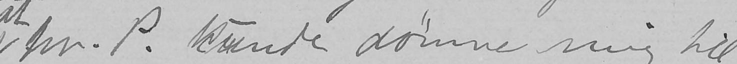hr. P. kunde dømme mig til
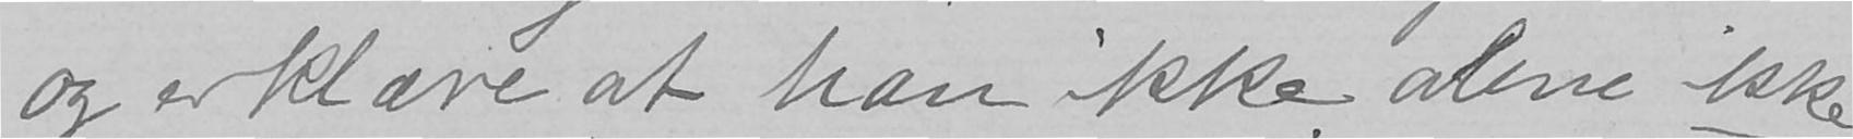og erklære at han ikke alene ikke
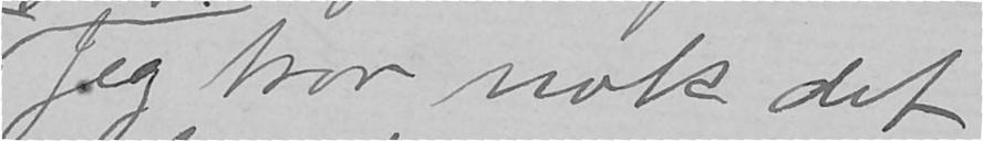Jeg tror nok det
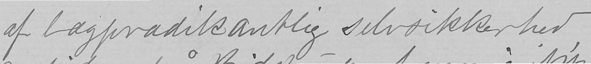af lægprædikantlig selvsikkerhed
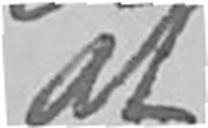at
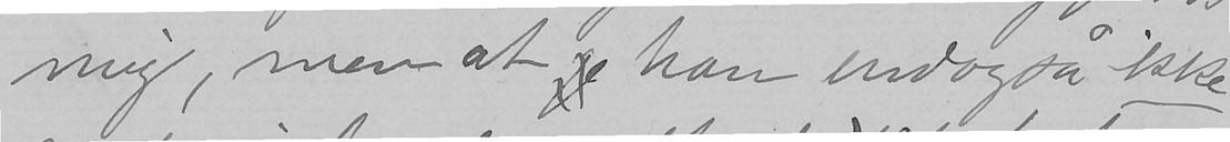mig, men at jeg han endogså ikke
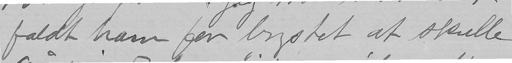faldt ham for brystet at skulle
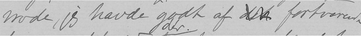trode, jeg havde godt af det fortvarende
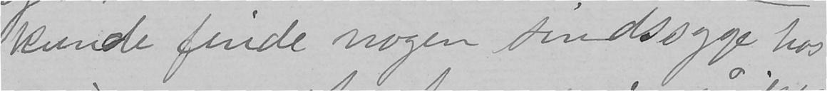kunde finde nogen sindssyge hos
